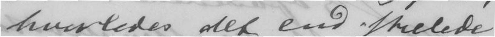hvorledes det end stillede
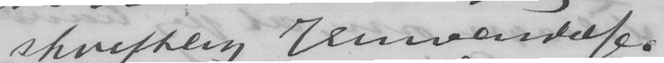skriftlig Henvendelse.
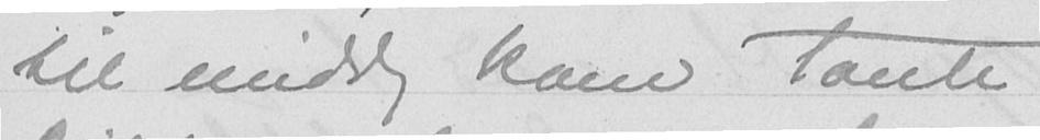til middag kom tante
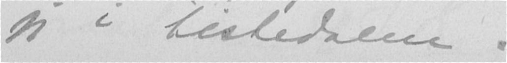jeg i Tistedalen.
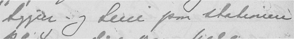Siggen og Leni paa stationen
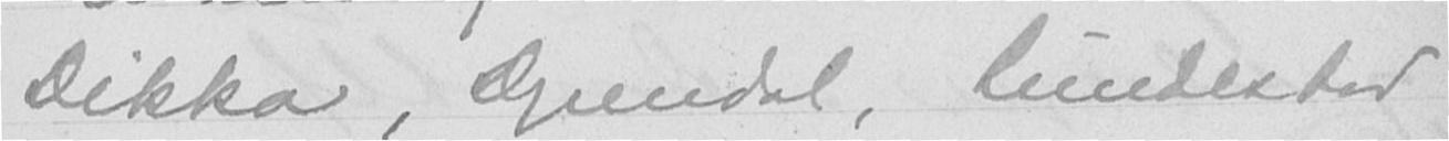Dikka, Dyrendal, Lundestad
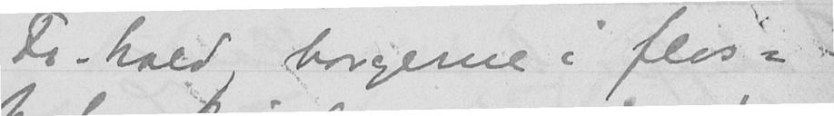Fr. hald borgerne i flos-
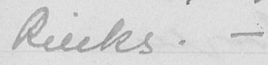Riecks. -
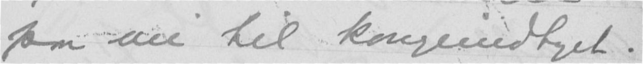paa vei til kongeindtoget.
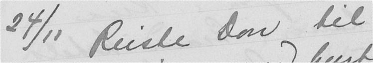24/11 Reiste Don til
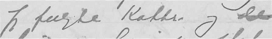Jeg fulgte Kathr. og de
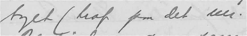toget (traf paa det mr.
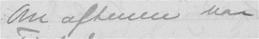Om aftenen var
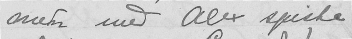men med Alex spiste
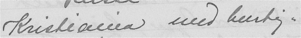Kristiania med hurtig-
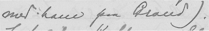med ham paa Grand).
